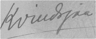Kvindsjaa
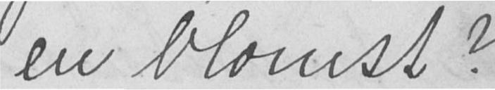en blomst?
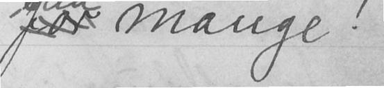for mange!
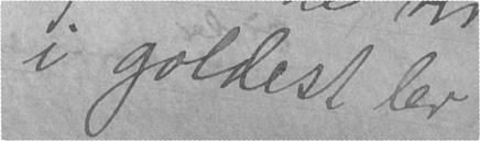i goldest ler
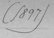(1897)
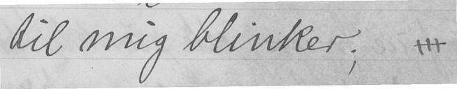til mig blinker; -
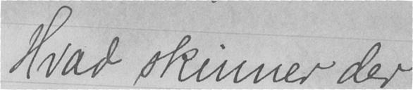Hvad skinner der
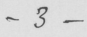- 3 -
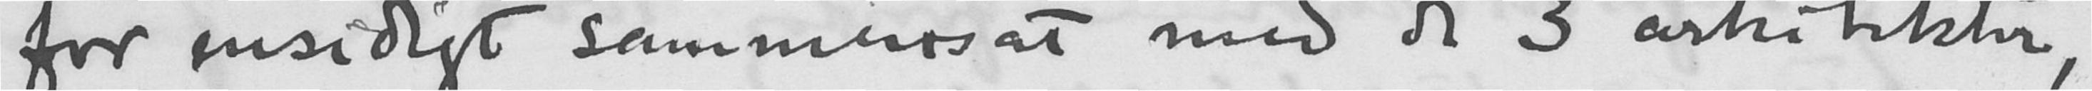for ensidigt sammensat med de 3 arkitekter,
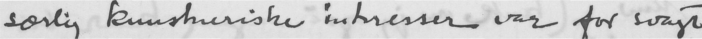særlig kunstneriske interesser var for svagt
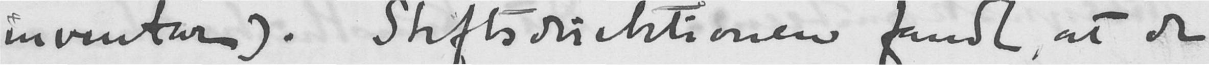inventar). Stiftsdirektionen fandt, at de
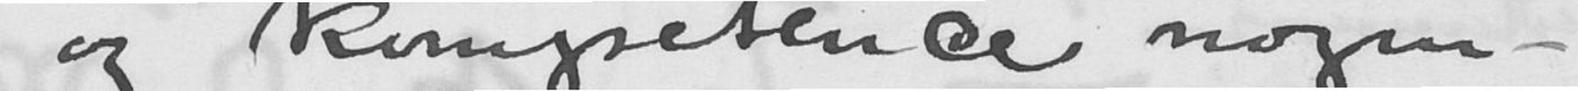og kompetence nogen-
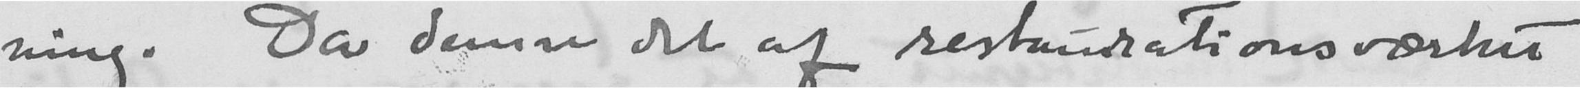ning. Da denne del af restaurationsværket
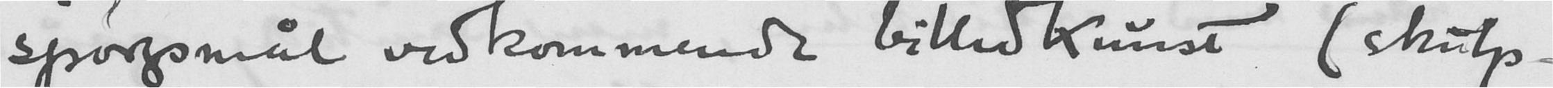spørgsmål vedkommende billedkunst (skulp-
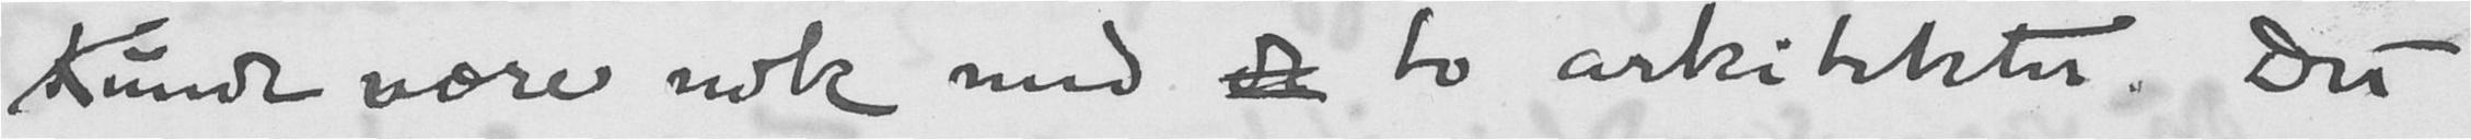kunde være nok med de to arkitekter. Det
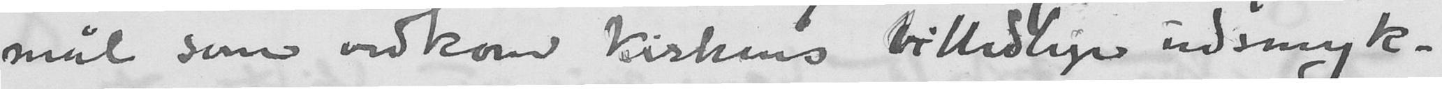mål som vedkom kirkens billedlige udsmyk-
